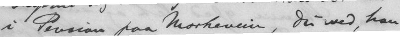i Pension paa Markeveien, du ved, han
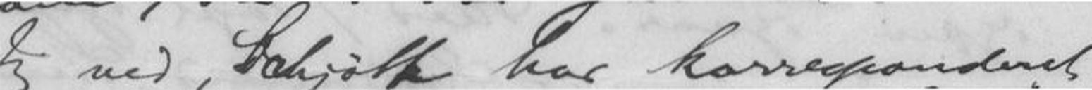Jeg ved, Schjøtt har korresponderet
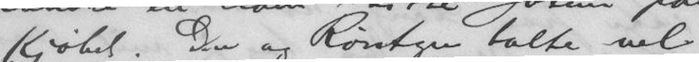Kjøbet. Du og Röntgen talte vel
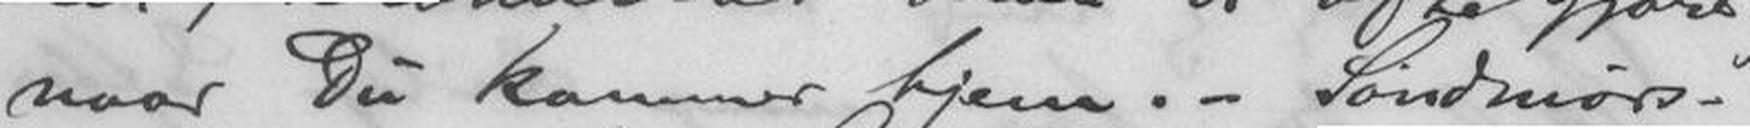naar Du kommer hjem. - Søndmørs-
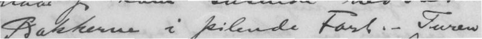Bakkerne i pilende Fart. - Turen
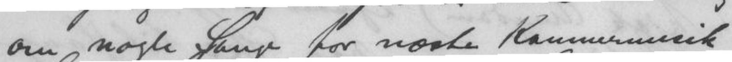om nogle Sange for næste Kammermusik-
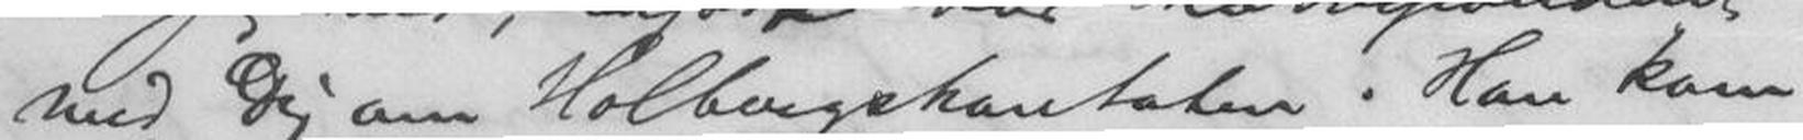med Dig om Holbergskantaten. Han kom
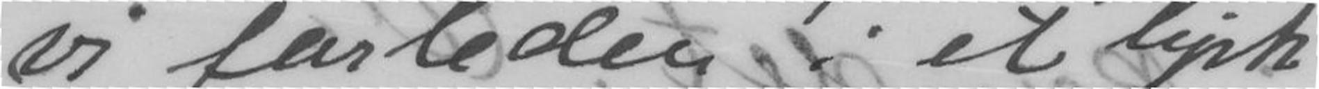vi forleden i et tysk
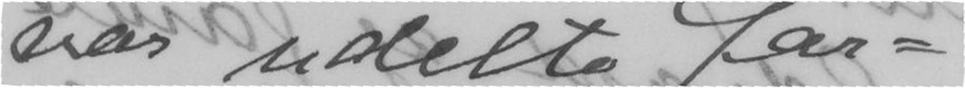vor udelt for=
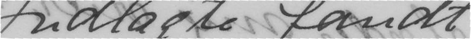Indlagt fandt
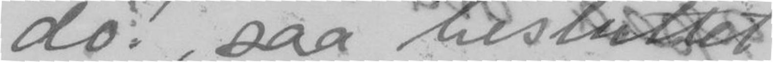dø, saa besluttet
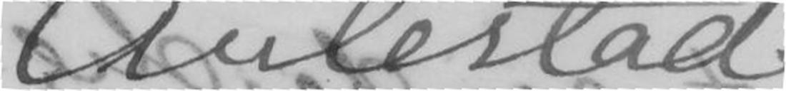Aulestad
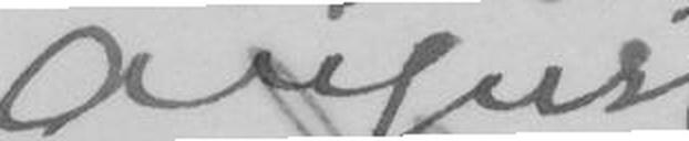August
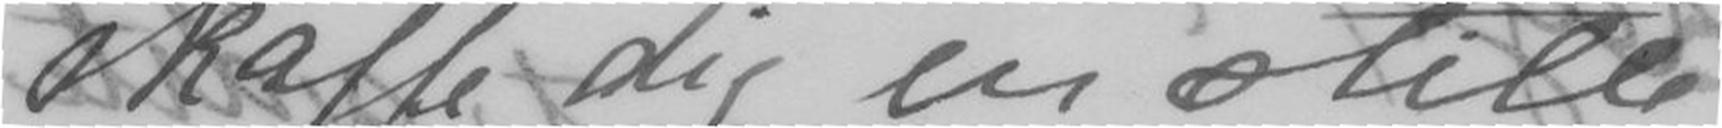skaffe dig en stille
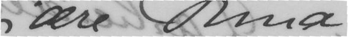Kjære Nina.
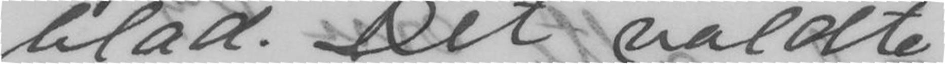blad. Det voldte
